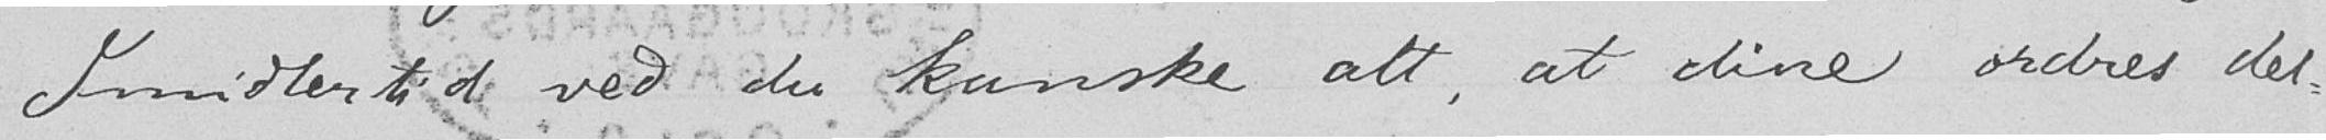Imidlertid ved du kanske alt, at dine ordres del-
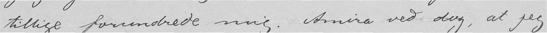tillige forundrede mig. Amira ved dog, at jeg
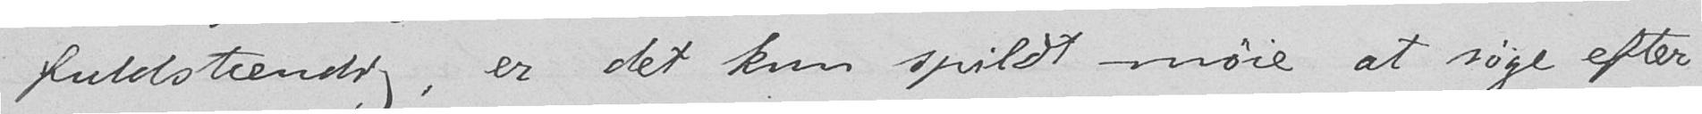fuldstændig, er det kun spildt møie at søge efter
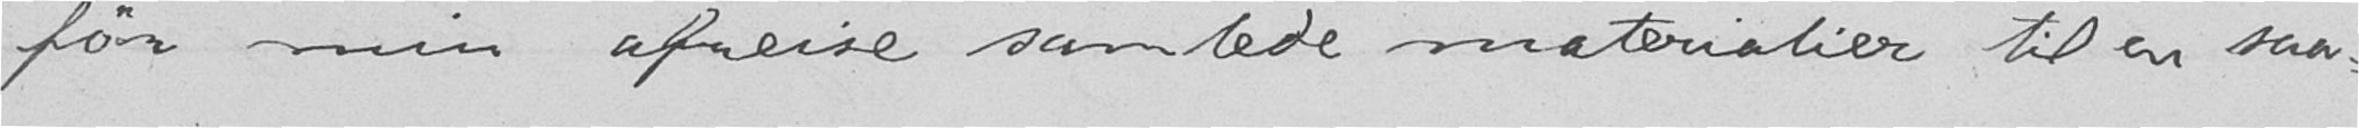før min afreise samlede materialier til en saa-
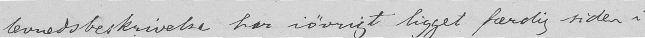levnedsbeskrivelse har iøvrigt ligget færdig siden i
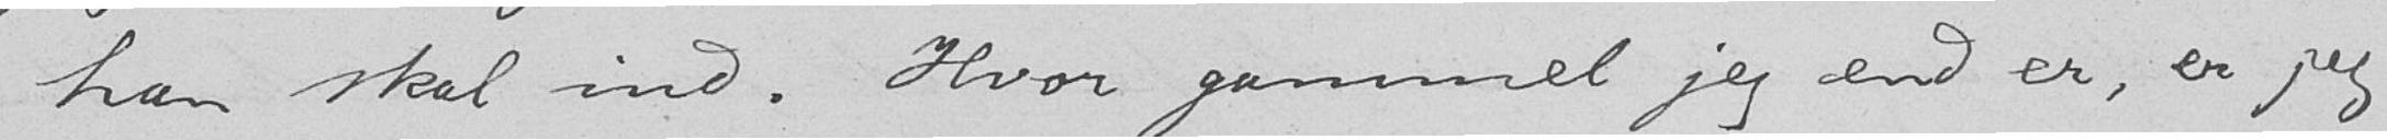han skal ind. Hvor gammel jeg end er, er jeg
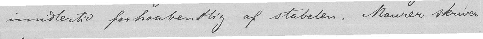imidlertid forhaabentlig af stabelen. Maurer skriver
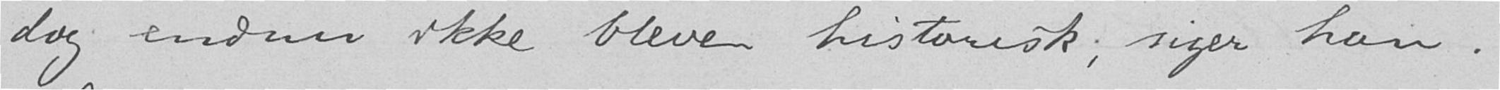dog endnu ikke bleven historisk, siger han.
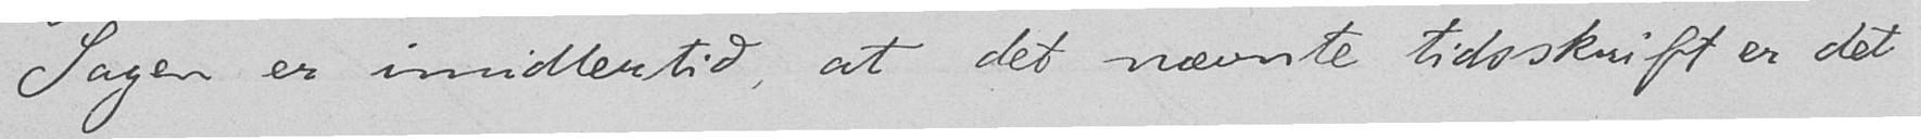Sagen er imidlertid, at det nævnte tidsskrift er det
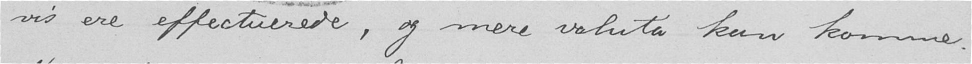vis ere effectuerede, og mere valuta kan komme.
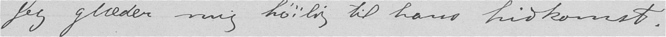Jeg glæder mig høilig til hans hidkomst.
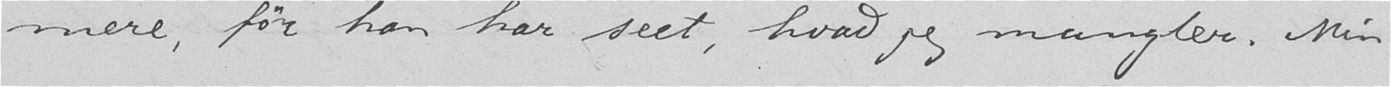mere, før han har seet, hvad jeg mangler. Min
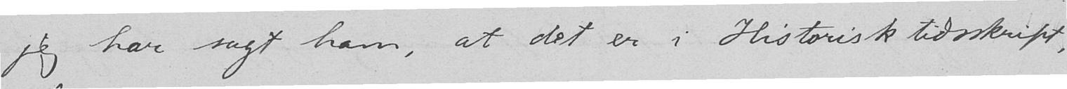jeg har sagt ham, at det er i Historisk tidsskrift,
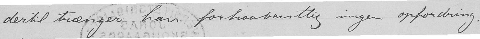dertil trænger han forhaabentlig ingen opfordring.
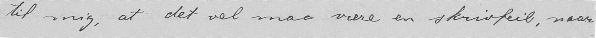til mig, at det vel maa være en skrivfeil, naar
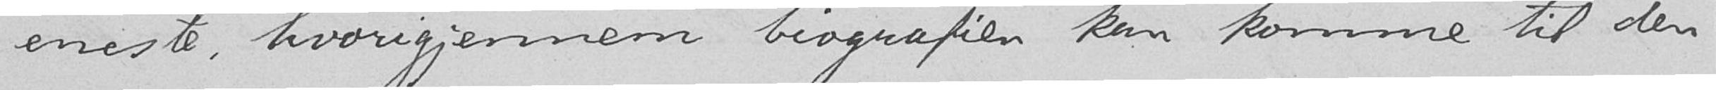eneste, hvorigjennem biografien kan komme til den
